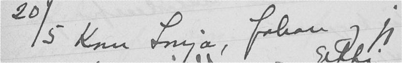20/5 Kom Sonja, Johan og jeg
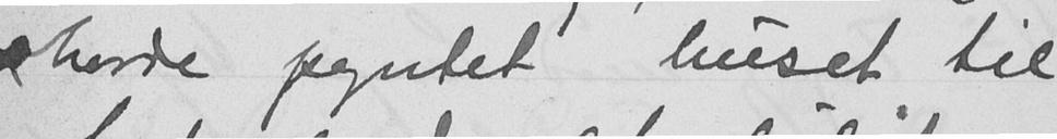havde pyntet huset til
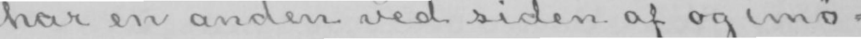har en anden ved siden af og imø-
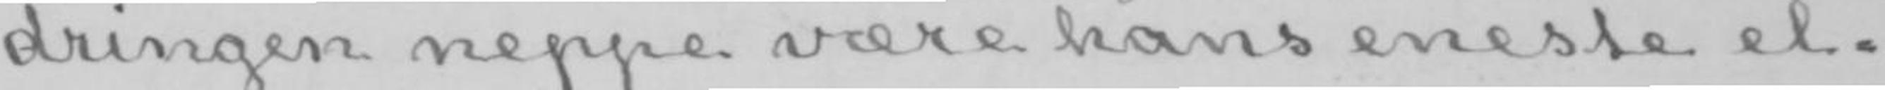dringen neppe være hans eneste el-
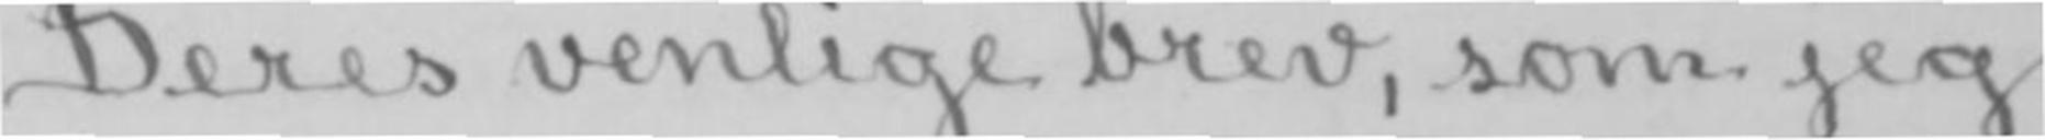Deres venlige brev, som jeg
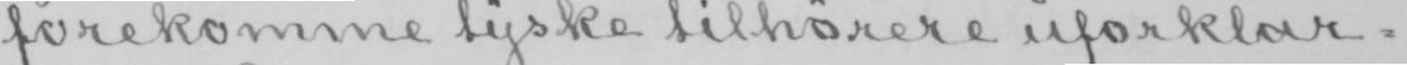forekomme tyske tilhørere uforklar-
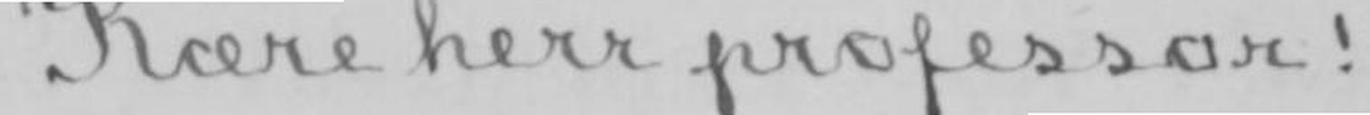Kære herr professor!
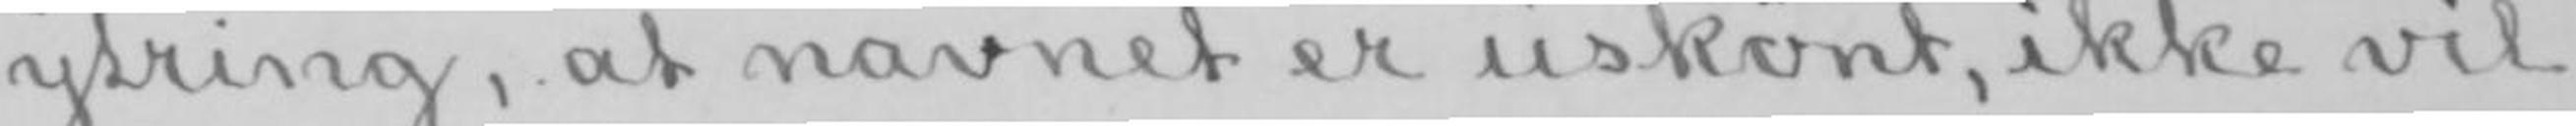ytring, at navnet er uskønt, ikke vil
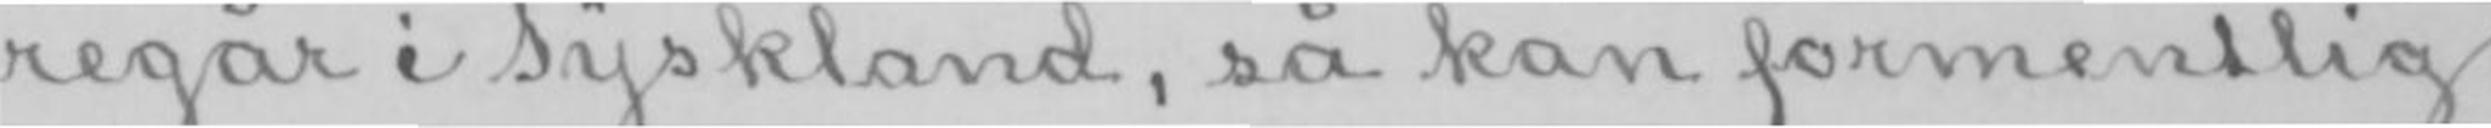regår i Tyskland, så kan formentlig
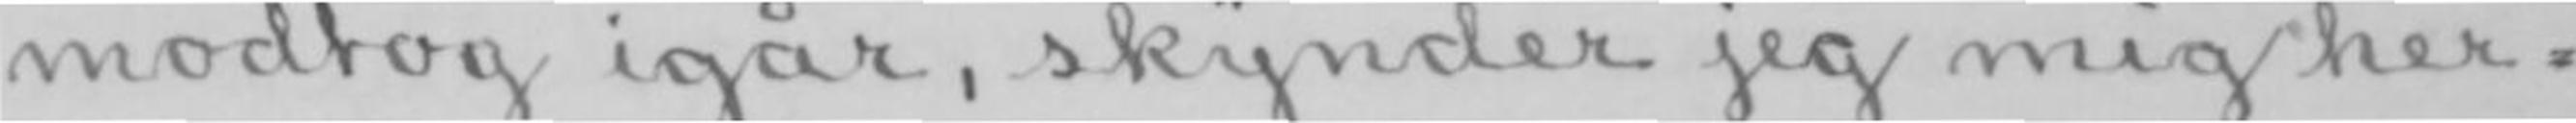modtog igår, skynder jeg mig her-
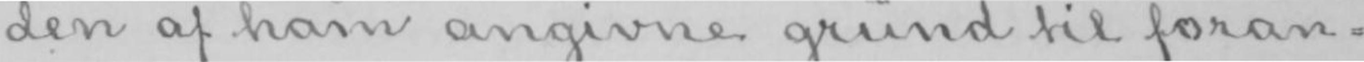den af ham angivne grund til foran-
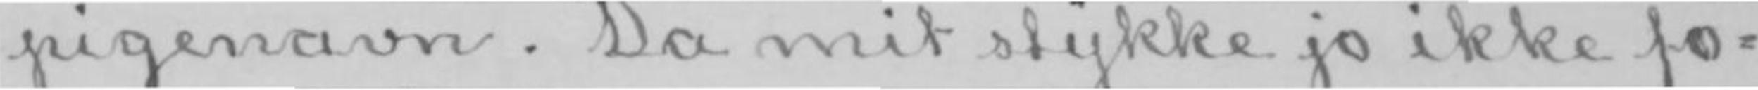pigenavn. Da mit stykke jo ikke fo-
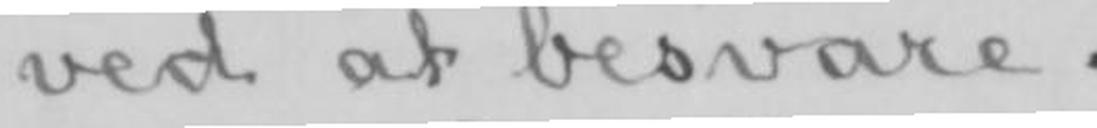ved at besvare.
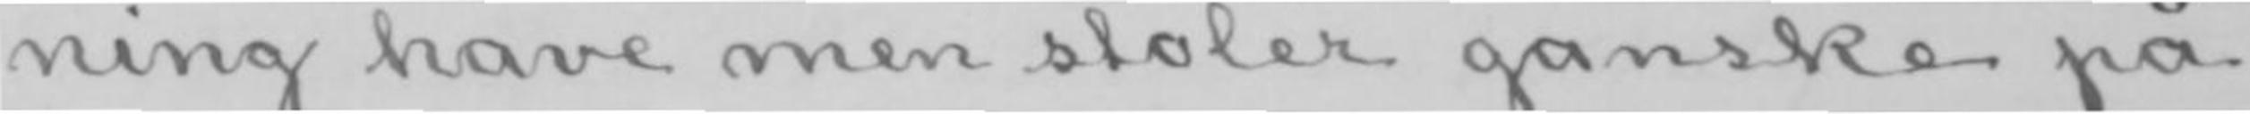ning have men stoler ganske på
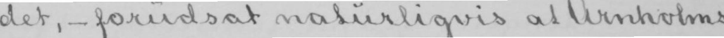det,- forudsat naturligvis at Arnholms
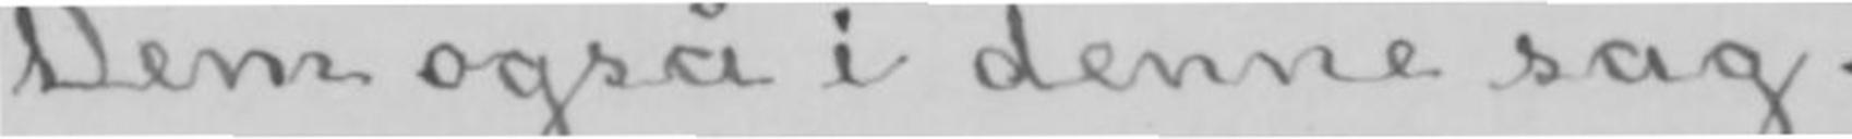Dem også i denne sag.
